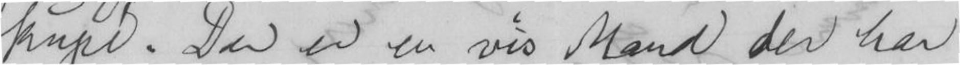kupe. Der er en vis Mand der har
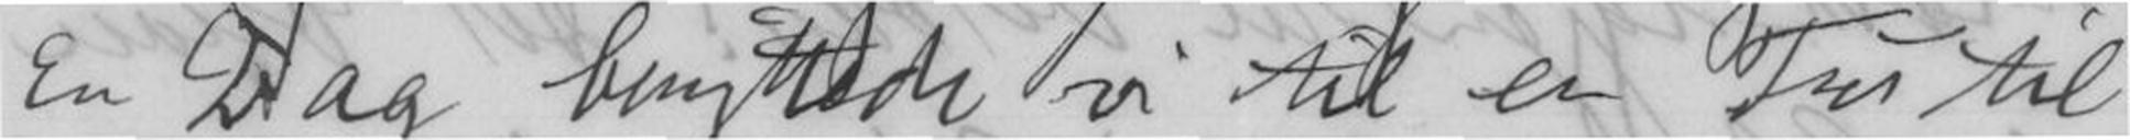En Dag benyttede vi til en Tur til
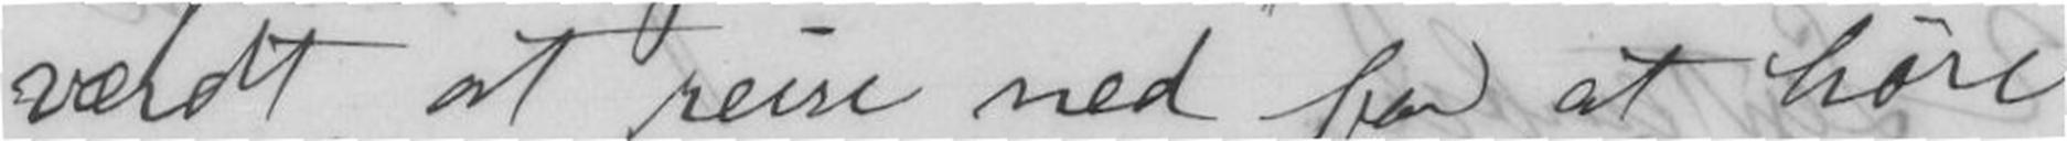værdt at reise ned for at høre.
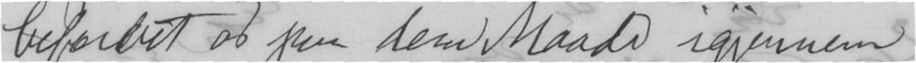befordret os paa den Maade igjennem
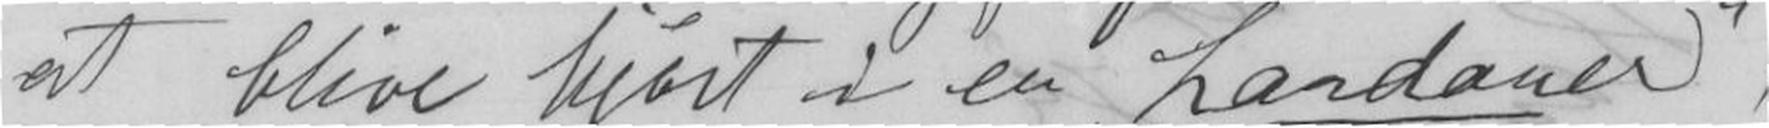at blive kjørt i en Landauer,
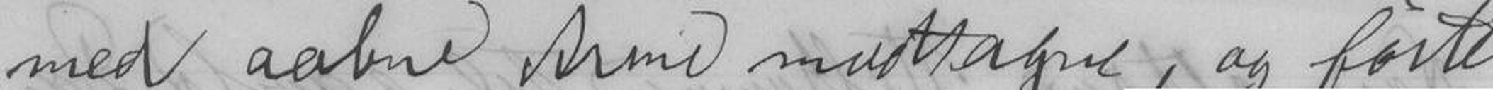med aabne Arme modtagne, og førte
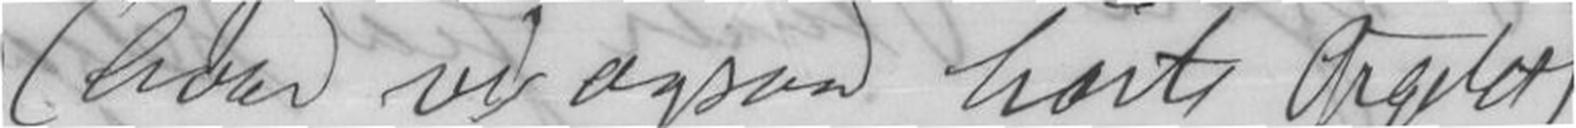(hvor vi ogsaa hørte Orgelet)
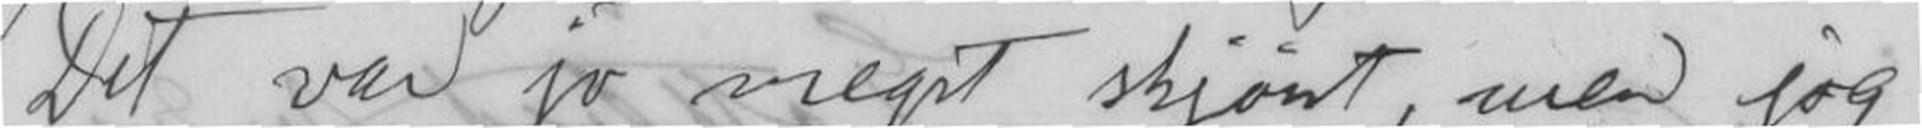Det var jo meget skjønt, men jeg
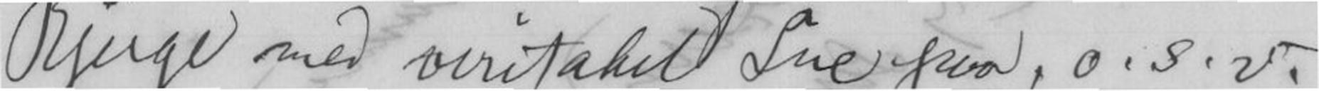Bjerge med overitakel Sne paa, o. s. v.
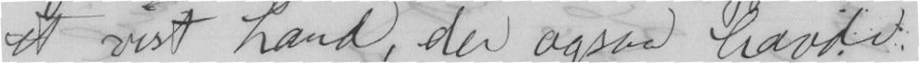et vist Land, der ogsaa havde
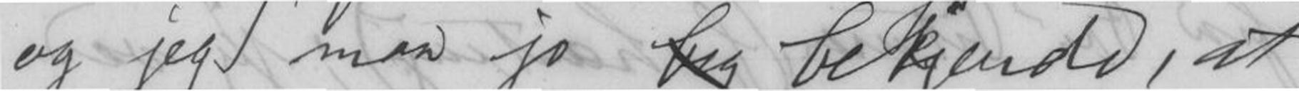og jeg maa jo bekjende, at
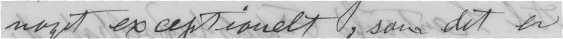noget exceptionelt; som det er
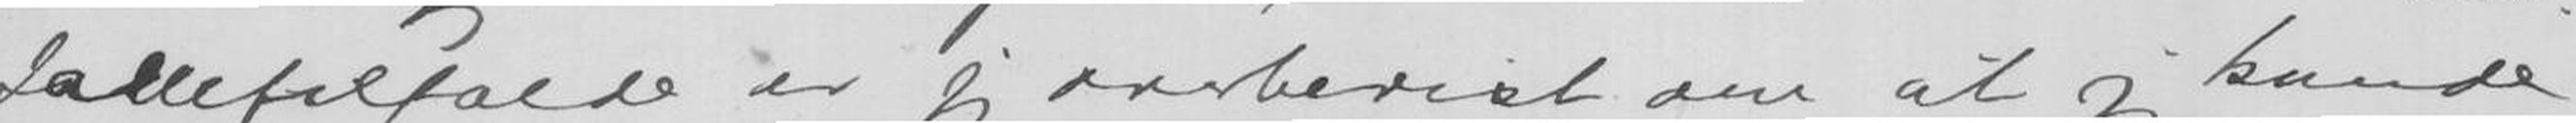Ialletilfælde er jeg overbevist om at jeg kunde
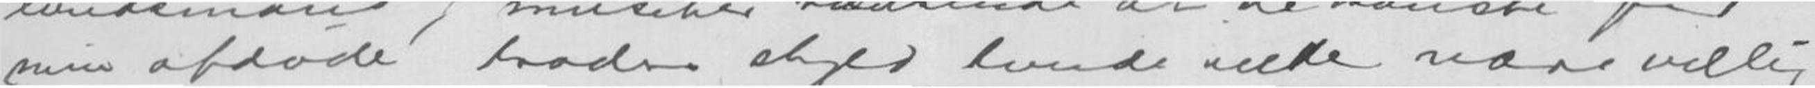min afdøde broder skyld kunde ville være villig
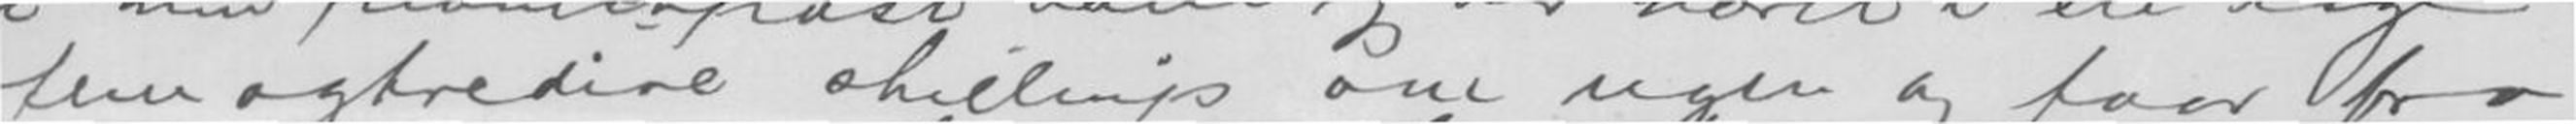fem og tredire shillings om ugen og faar fra
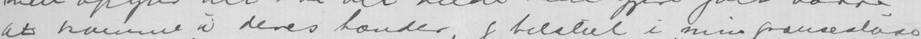at komme i deres hænder, og tilslut i min grenseløse
